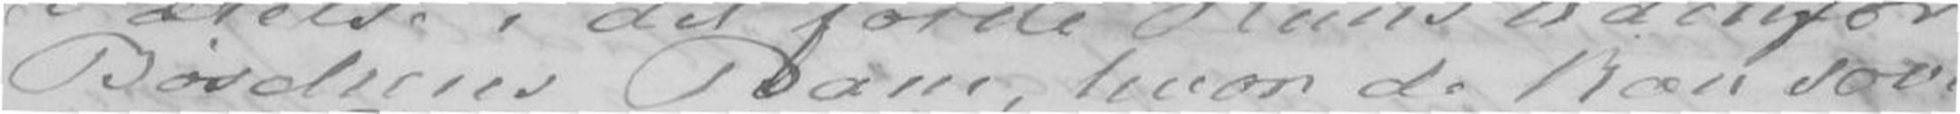Bøschens Bane, hvor de kan sove
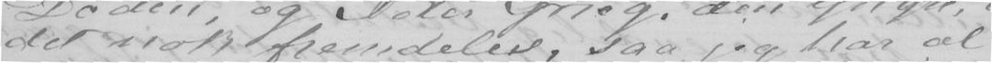det nok fremdeles, saa jeg har al
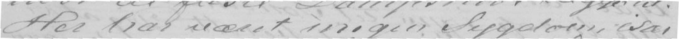Her har været megen Sygdom, især
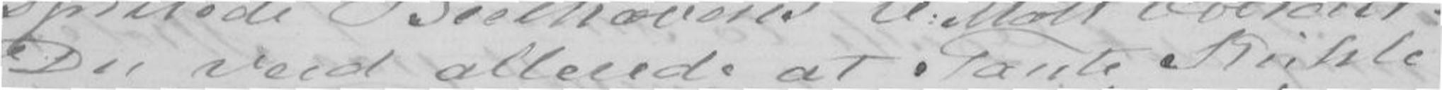Du veed allerede at Tante Kühle
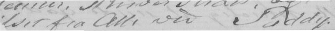lset fra Alle ved Paddy.
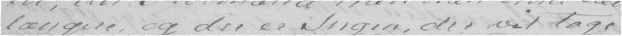længere, og der er Ingen der vil tage
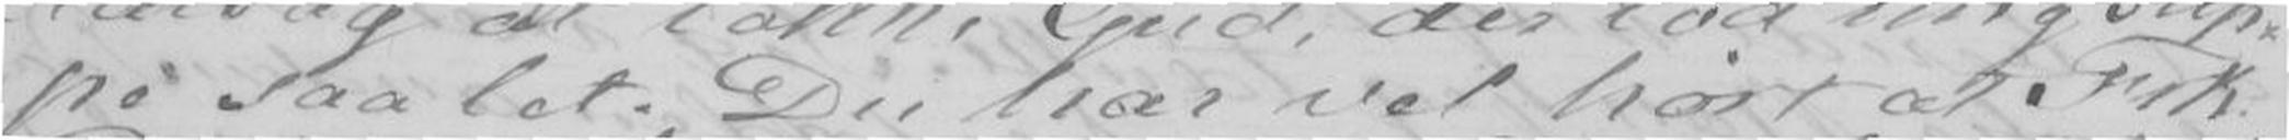pe saa let. Du har vel hørt at Frk
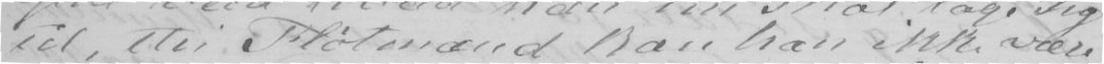til, thi Fløtemænd kan han ikke være
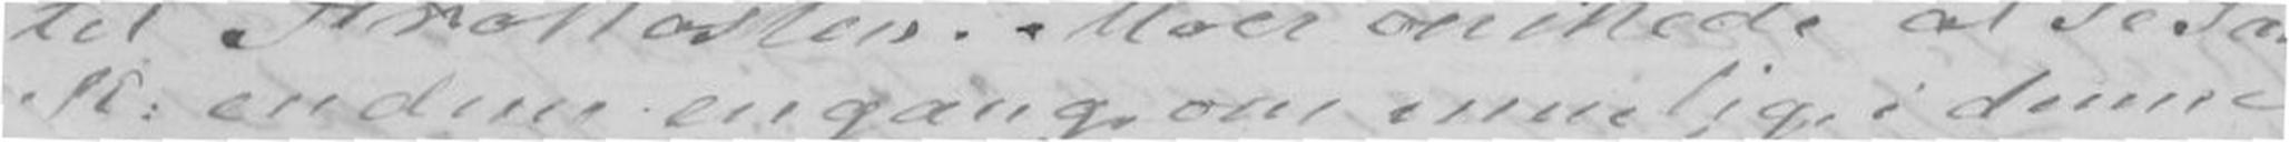K: endnu engang om muelig i denne
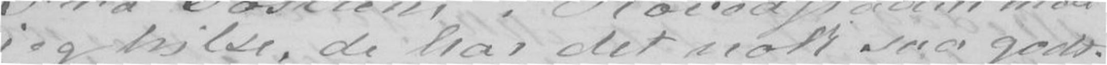jeg hilse, de har det nok saa godt.
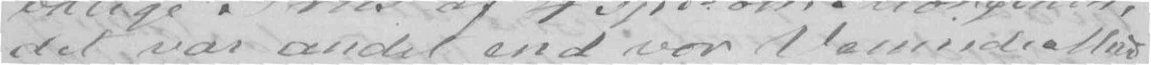det var andet end vor Veninde Mad
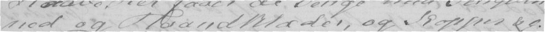ned og Haandklæder, og Kopper ogc
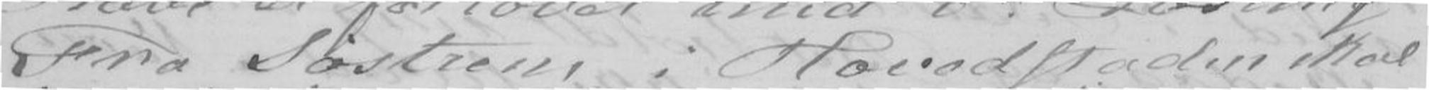Fra Søstrene i Hovedstaden skal
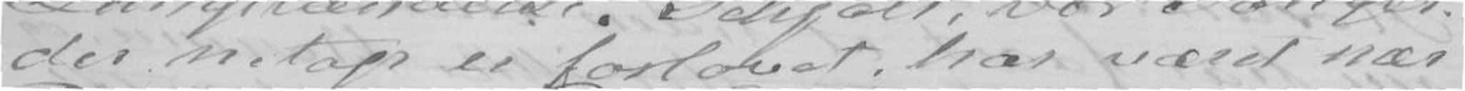der netop er forlovet, har været nær
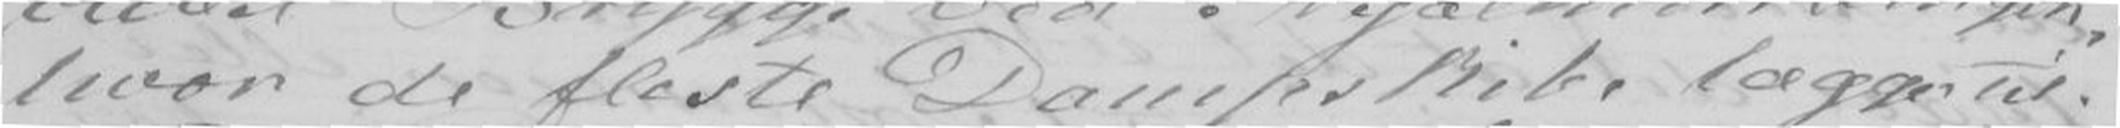hvor de fleste Dampskibe lægger til.
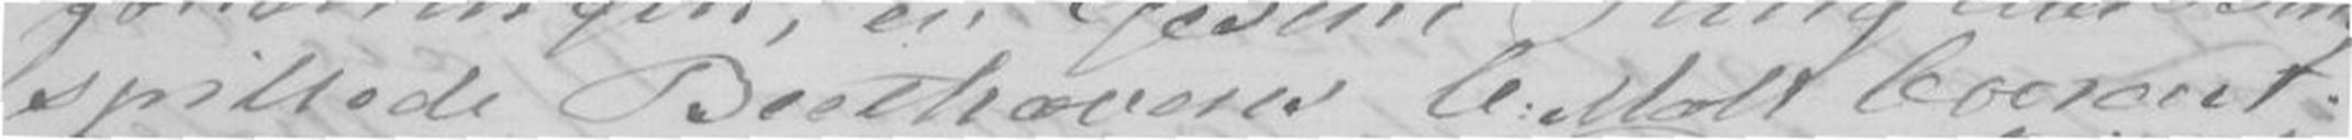spillede Beethovens C:Moll Concert.
